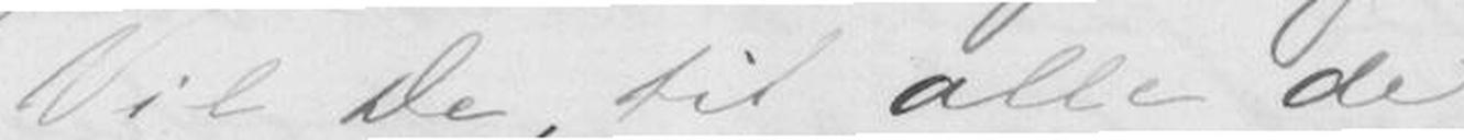Vil De, til alle de
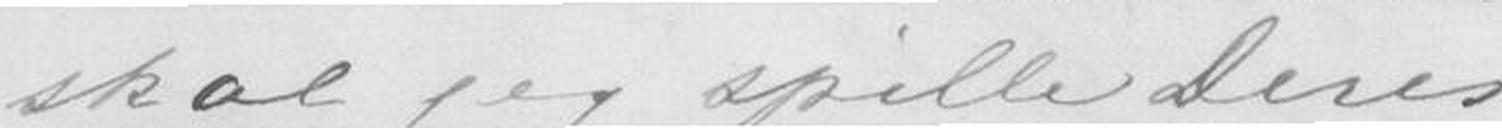skal jeg spille Deres
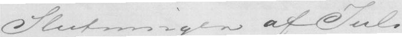slutningen af Juli
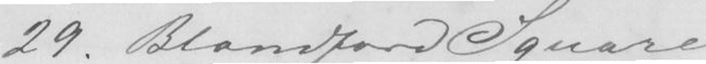29 Blandford Square
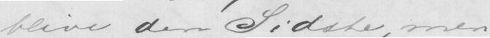blive den sidste, men
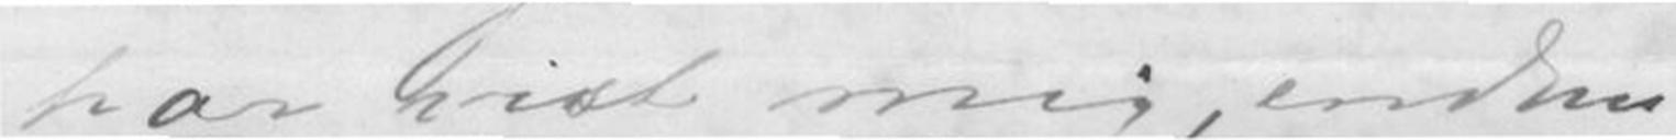har vist mig, endnu
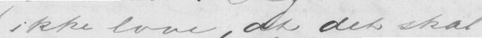ikke love, at det skal
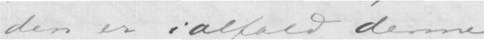den er i alfald denne
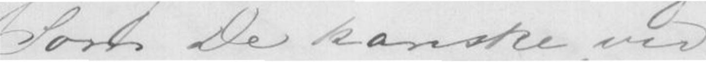Som De kanske ved,
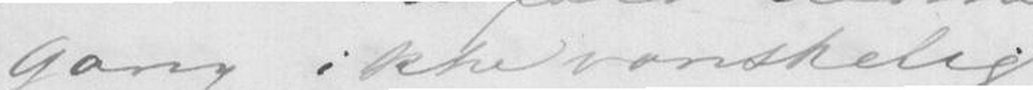Gang ikke vanskelig.
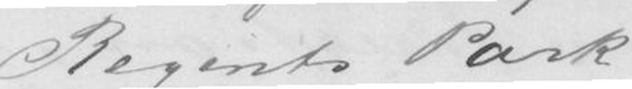Regents Park
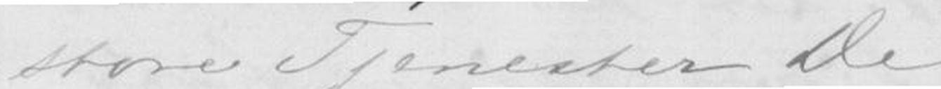store Tjenester De
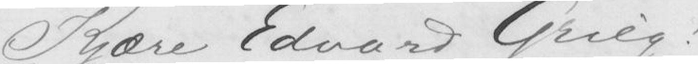Kjære Edvard Grieg!
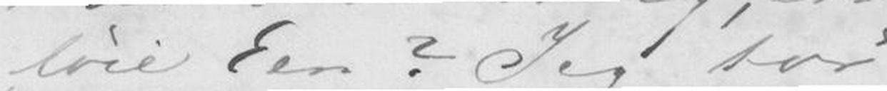føie Een? Jeg tør
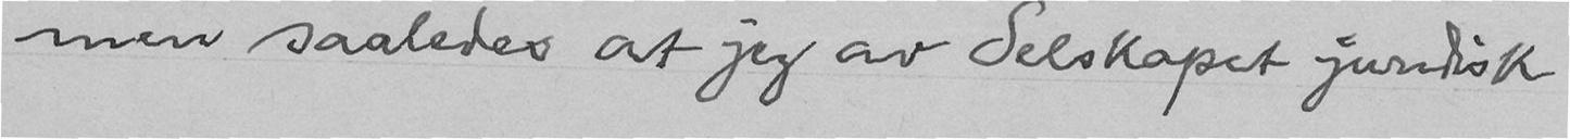men saaledes at jeg av Selskapet juridisk
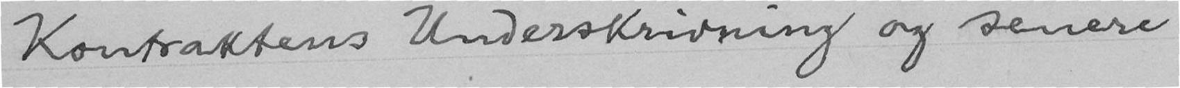Kontraktens Underskrivning og senere
